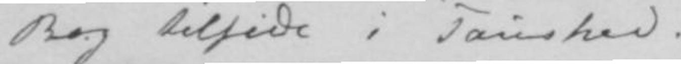Bog tilside i Taushed.
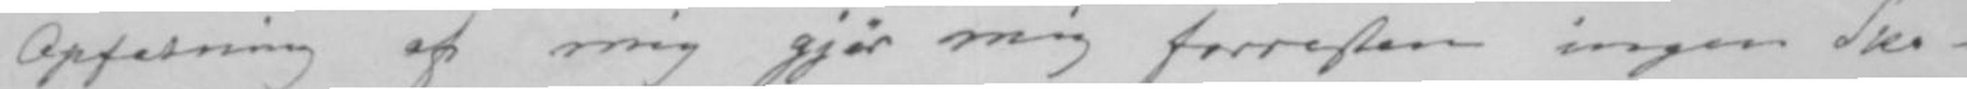Opfatning af mig gjør mig forresten ingen Ska-
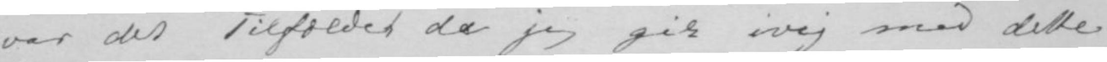var det Tilfældet da jeg gik ivej med dette
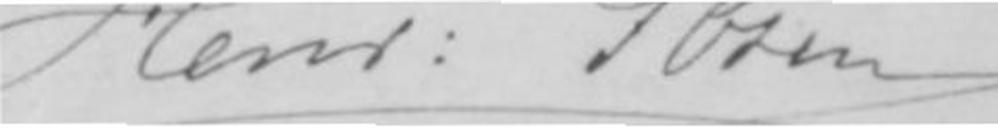Henr: Ibsen
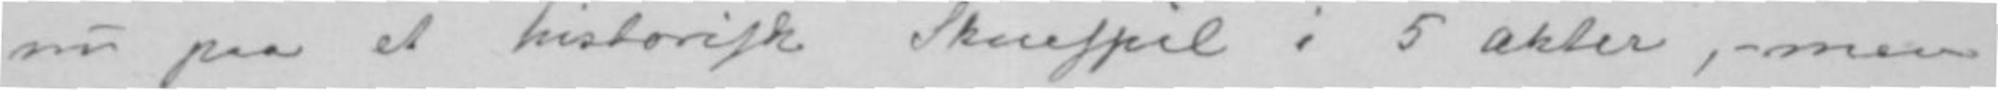nu paa et historisk Skuespil i 5 Akter,- men
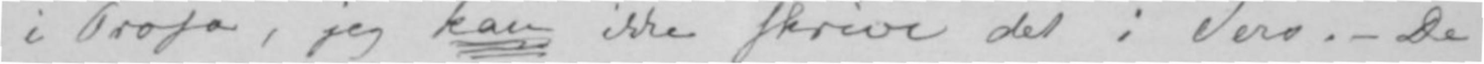i Prosa, jeg kan ikke skrive det i Vers.- De
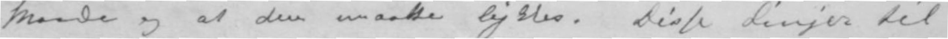Maade og at den maatte lykkes. Disse Linjer til
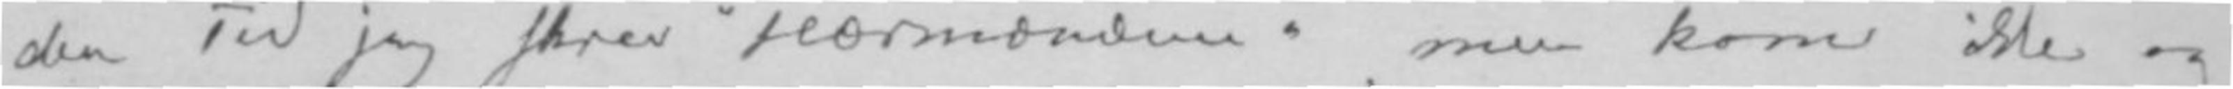den Tid jeg skrev «Hærmændene» men kom ikke og
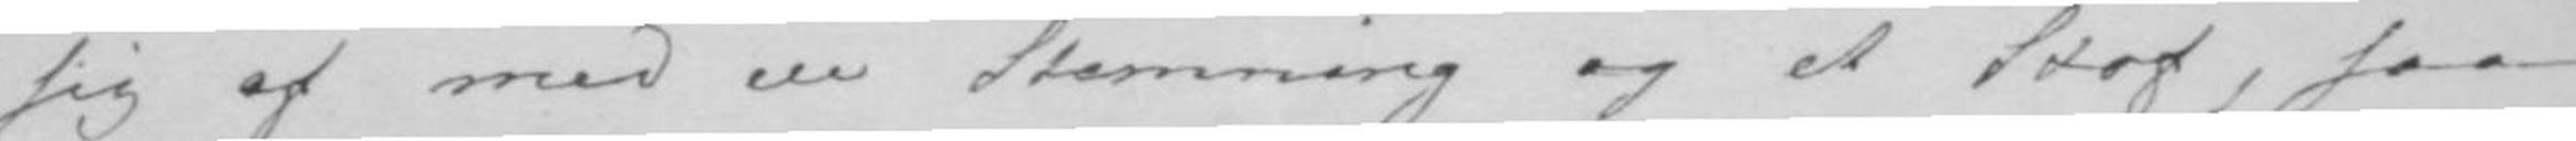sig af med en Stemning og et Stof, saa
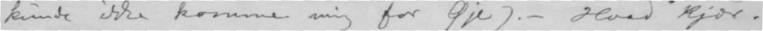kunde ikke komme mig for Øje).- Hvad «Kjær-
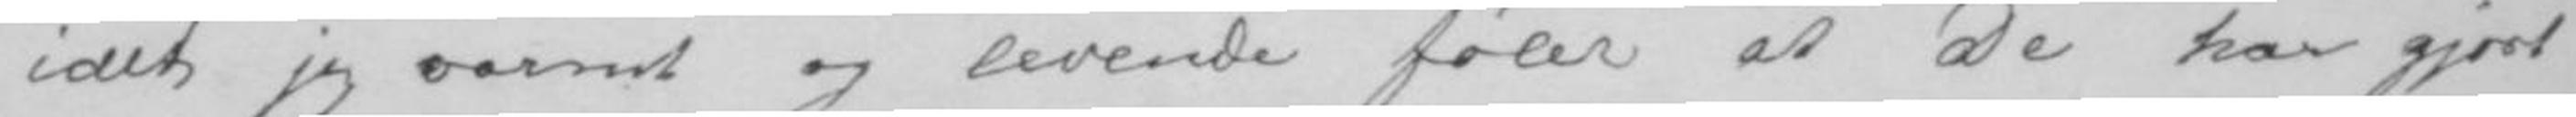idet jeg varmt og levende føler at De har gjort
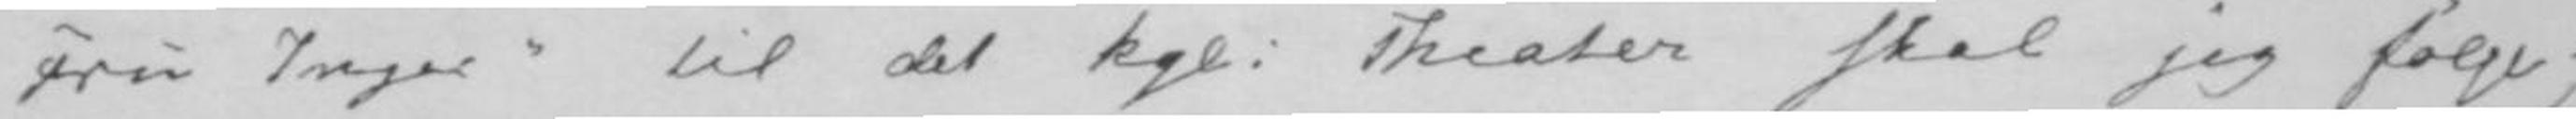«Fru Inger» til det kgl: Theater skal jeg følge;
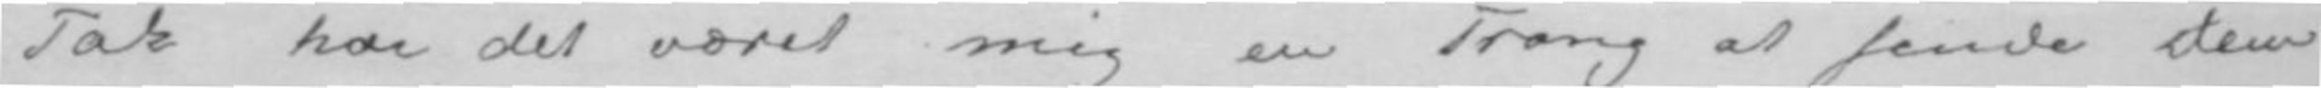Tak har det været mig en Trang at sende Dem
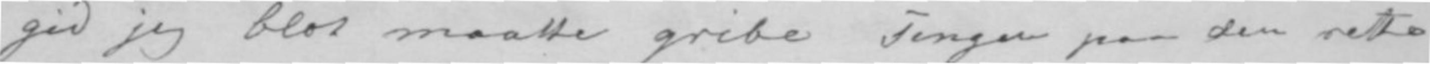gid jeg blot maatte gribe Tingen paa den rette
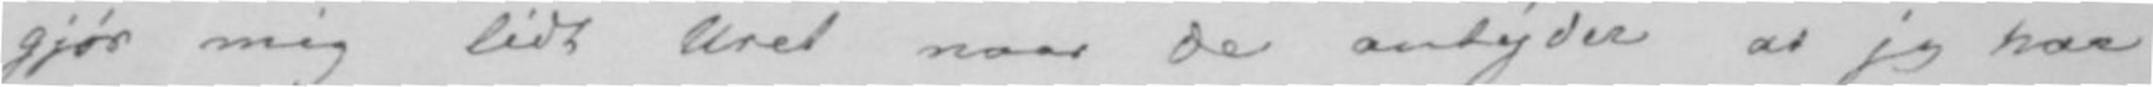gjør mig lidt Uret naar De antyder at jeg har
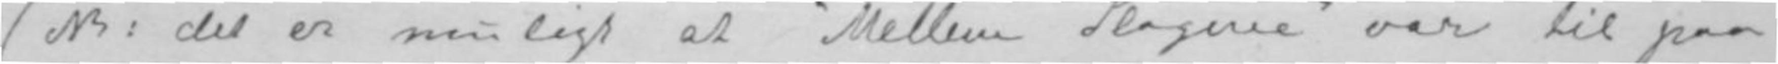(NB: det er muligt at «Mellem Slagene» var til paa
